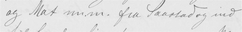og Mat m.m. fra Saastad og ind
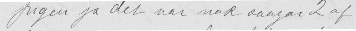pigen ja det var nok saagar 2 af
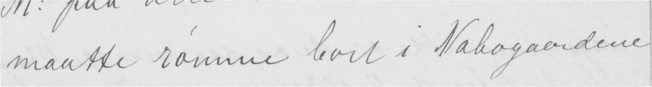maatte rømme bort i Nabogaardene
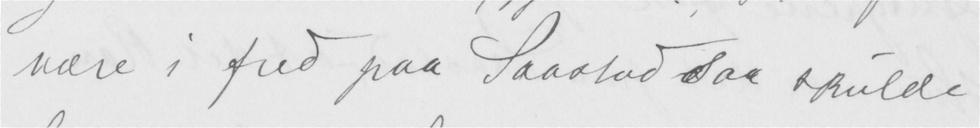være i fred paa Saastad saa skulde
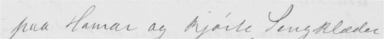paa Hamar og kjørte Sengklæder
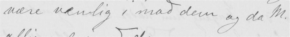være venlig i mod dem og da M.
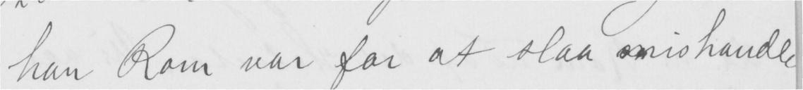han kom var for at slaa mishandle
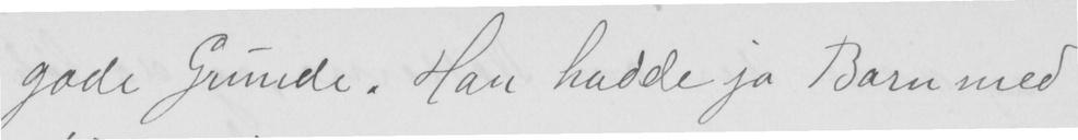gode Grunde. Han hadde jo Barn med
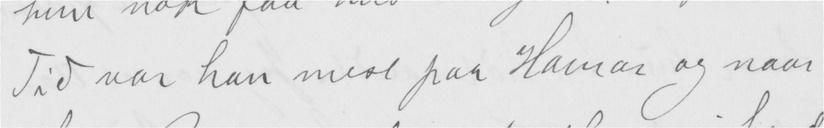Tid var han mest paa Hamar og naar
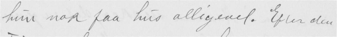hun nok faa hus alligivel. Efter den
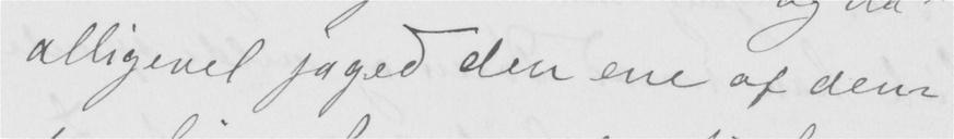alligevel jaged den ene af dem
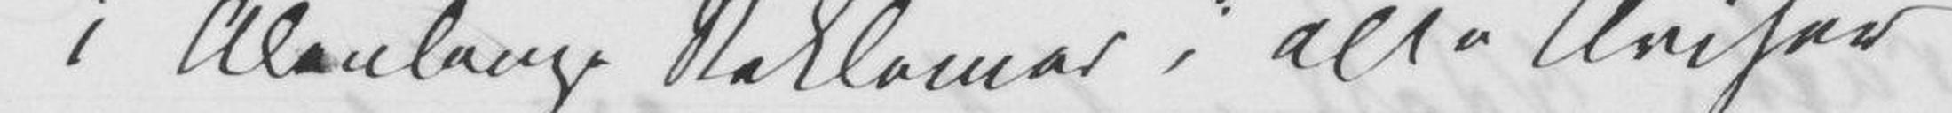i Alenlange Reklamer i alle Aviser
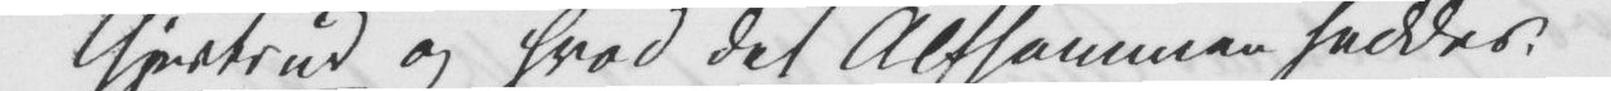Gjertrud og hvad det Altsammen hedder.
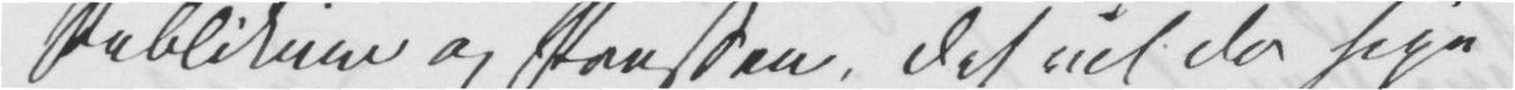Publikum og Pressen, det vil da sige
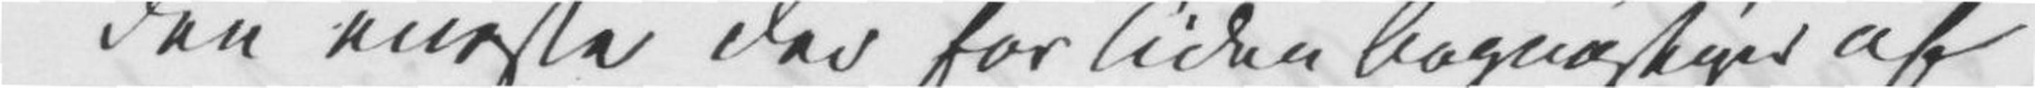Den eneste der for Tiden begunstiges af
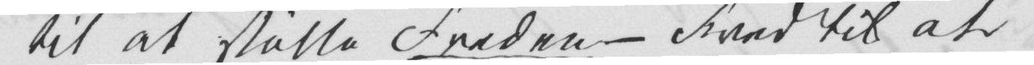til at støtte Freden – Fred til at
